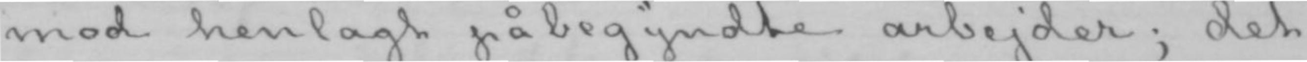mod henlagt påbegyndte arbejder; det
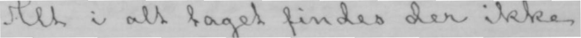Alt i alt taget findes der ikke
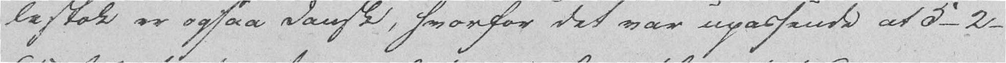lestok er ogsaa dansk, hvorfor det var upassende at 5-2-
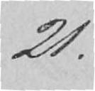21.
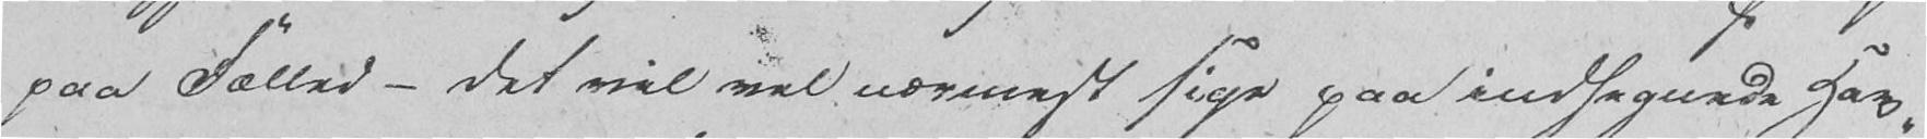paa Fælled - det vil vel nærmest sige paa indhegnede Hav-
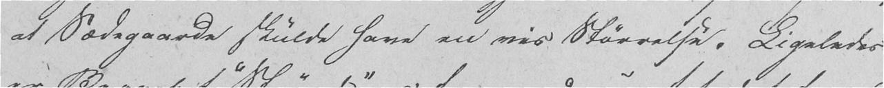at Sædegaarde skulde have en vis Størrelse. Ligeledes
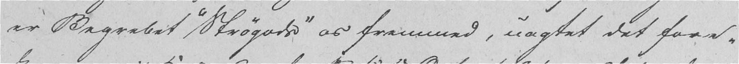er Begrebet "Strøgods" os fremmed, uagtet det fore-
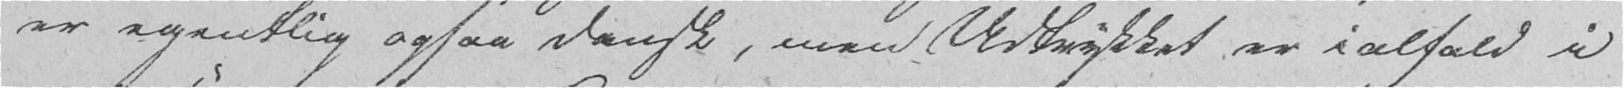er egentlig ogsaa dansk, men Udtrykket er i alfald i
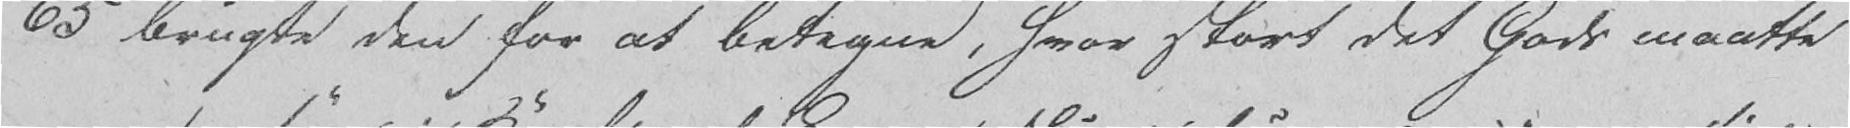65 brugte den for at betegne, hvor stort det Gods maatte
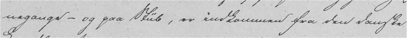negange - og paa Stub, er indkommen fra den danske
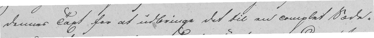dennes Taxt for at udbringe det til en complet Sæde-
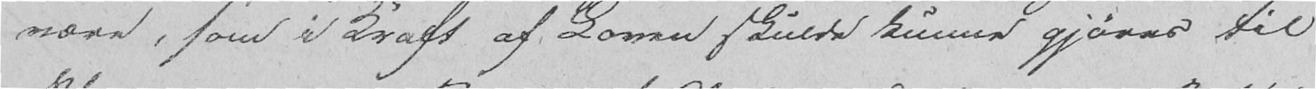være, som i Kraft af Loven skulde kunne gjøres til
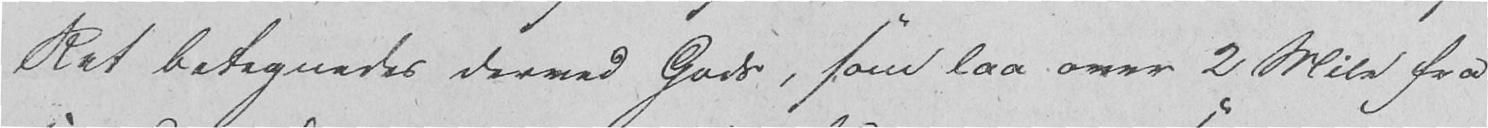Ret betegnedes derved Gods, som laa over 2 Mile fra
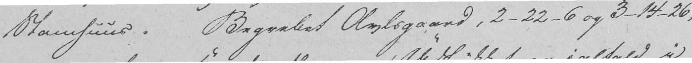Stamhuus. Begrebet Avlsgaard, 2-22-6 og 3-14-26,
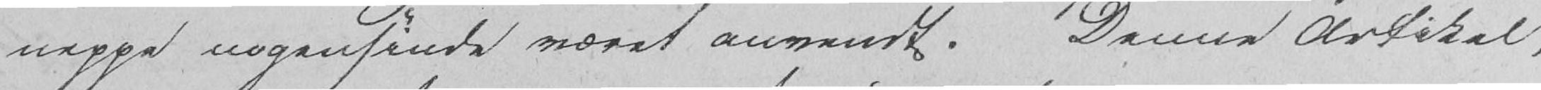neppe nogensinde været anvendt. Denne Artikel,
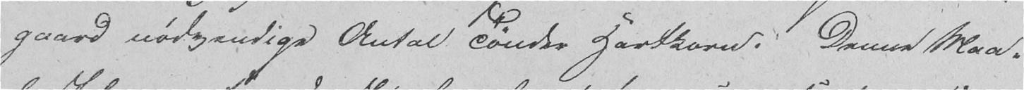gaard nødvendige Antal Tønder Hartkorn. Denne Maa-
